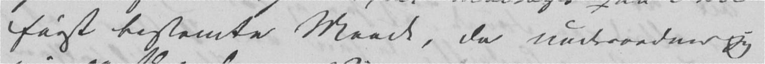først bestemte Maade, da underordner jeg
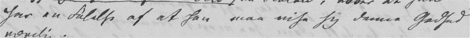har en Følelse af at han maa vise sig denne Godhed
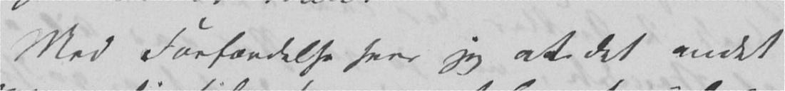Med Forfærdelse seer jeg at det andet
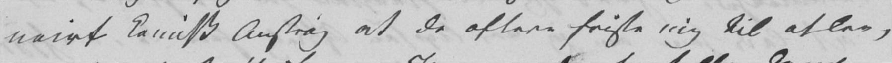naivt komisk Anstrøg at de oftere friste mig til at lee,
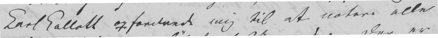Carl Collett opfordrede mig til at notere alle
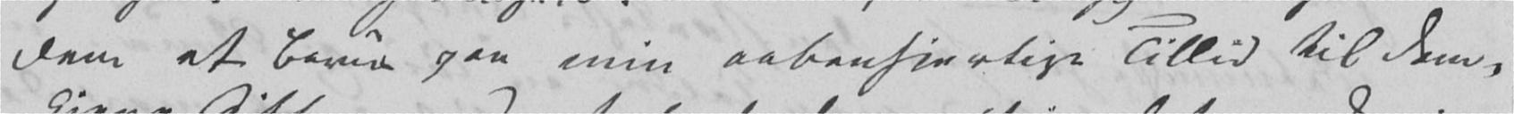Dem et Beviis paa min aabenhjertige Tillid til Dem,
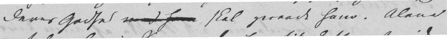Deres Godhed mod ham skal geraade ham. Alene
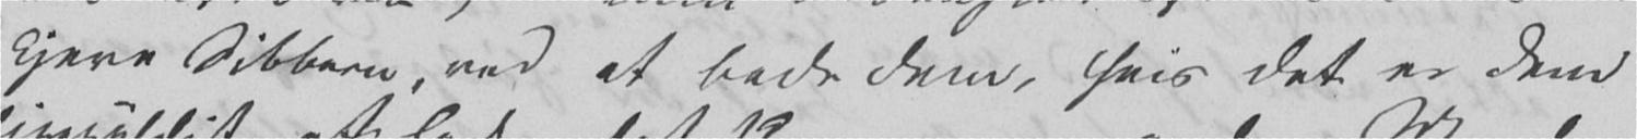kjere Sibbern, ved at bede Dem, hvis det er Dem
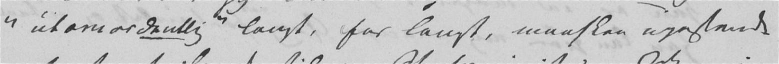«utomordentlig» langt, for langt, maaskee upassende
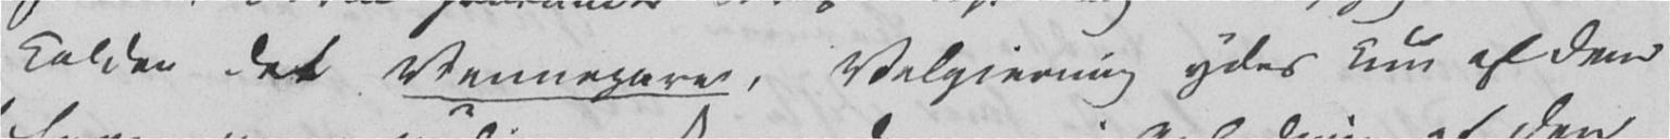kalde det Vennegave, Velgjerning ydes kun af dem
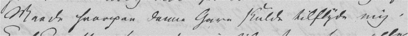Maade hvorpaa denne Gave skulde tilflyde mig.
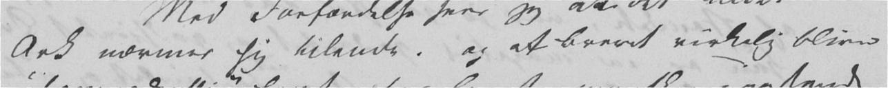Ark nærmer sig tilende. og at Brevet virkelig bliver
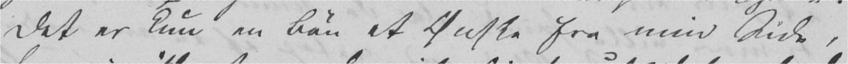Det er kun en Bøn et Ønske fra min Side,
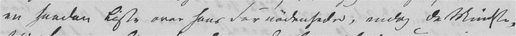en saadan Liste over hans Fornødenheder, endog de Mindste,
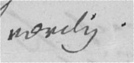værdig.
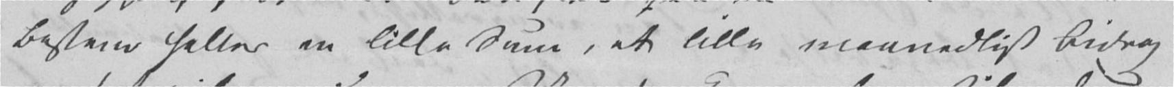bestem heller en lille Sum, et lille maanedligt Bidrag
